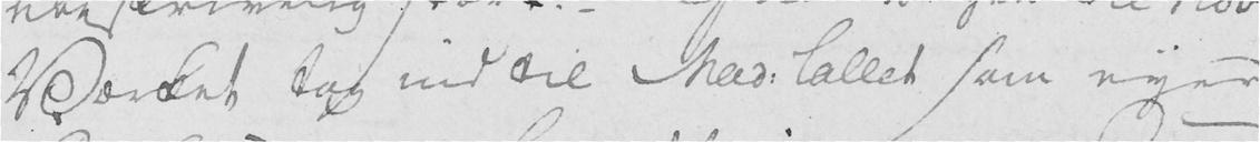Værket tog ind til Mad. Collet som eyer
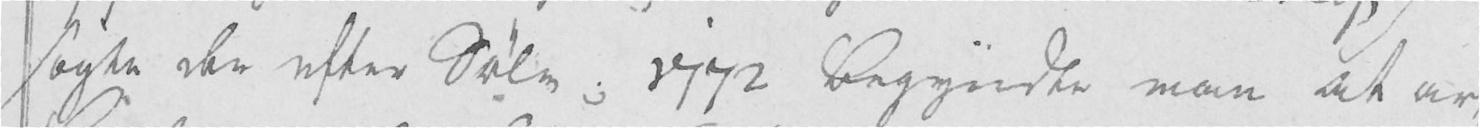søgte der efter Sølv; 1772 begyndte man at ar-
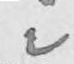i
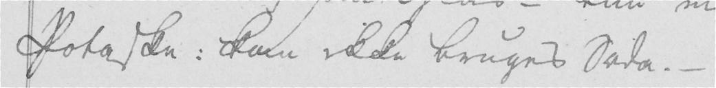Potaske: kan ikke bruges Soda. -
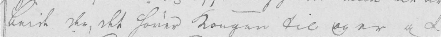beide der, det hører Kongen til og er af
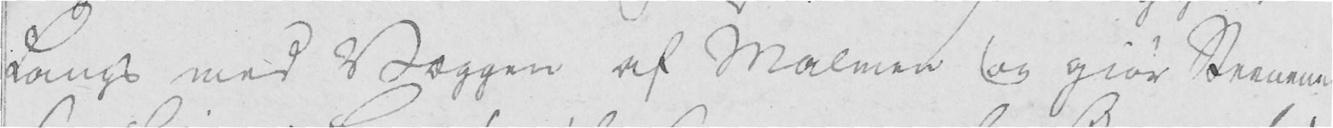Langs med Væggen af Malmen og giør Steenene
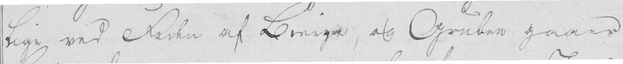lige ved Foden af Bierge, og Gruben gaaer
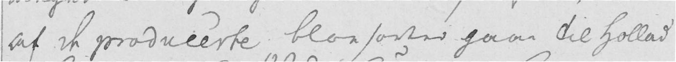af de producerte blaa sorter gaar til Holland
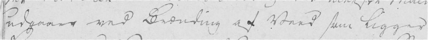udgaaer ved Brænding af Veed som ligger
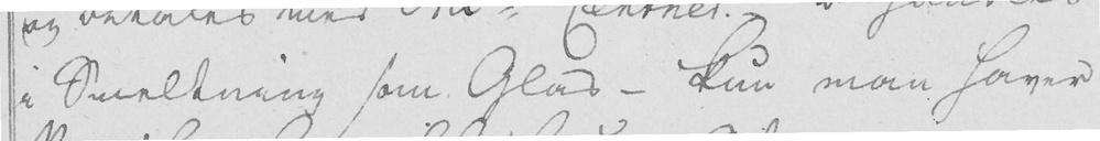i Smeltning som Glas - kun man haver
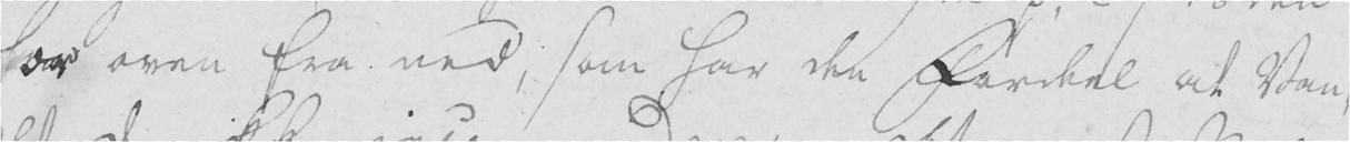for oven fra ned, som har den Fordeel at Van-
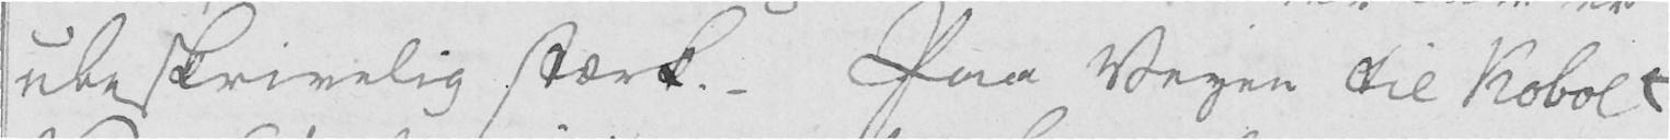ubeskrivelig stærk. - Paa Veyen til Kobolt
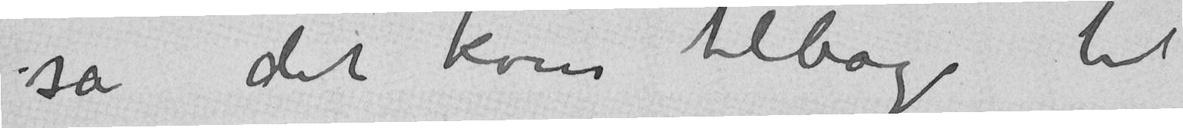så det kom tilbage til
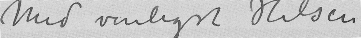Med venligst Hilsen
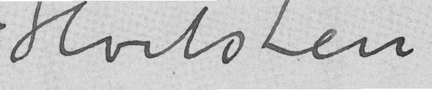Hvitsten
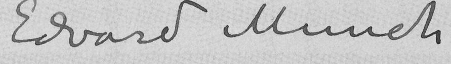Edvard Munch
